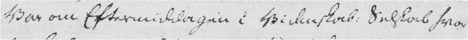Var om Eftermiddagen i Videnskab. Selskab hvor-
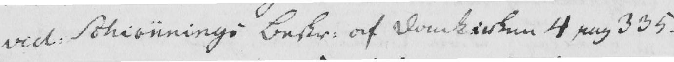vict. Schiønnings beskr. af Domkirken 4 pag 335.
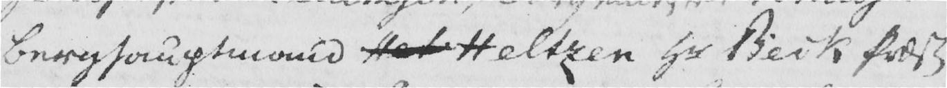Berghauptmand  Heltzen Hr Beck Præst
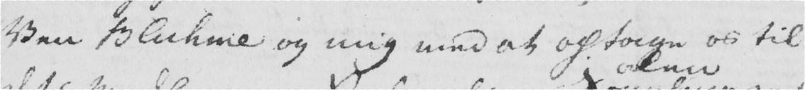Ven Bluhme og mig med at optage os til
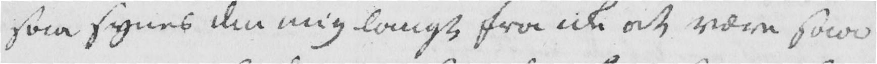som synes den mig langt fra icke at være saa
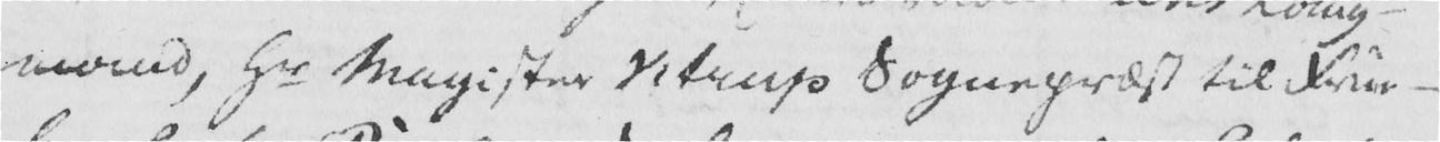mand, Hr Magister Vitrup Sognepræst til Frue-
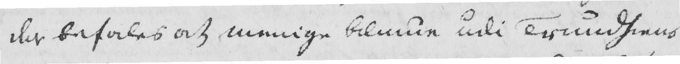der befales at menige Almue udi Trundhiems
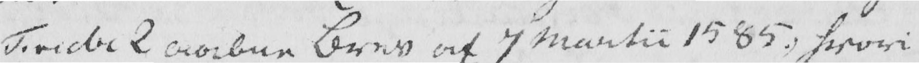Fredr 2 aabne Brev af 7 Martii 1585, hvori
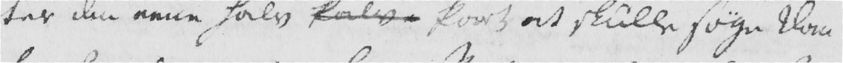ter den eene halve  Part at skulle søge Dom-
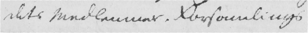dets Medlemmer. Forsamlings
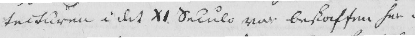tecturen i det XI Seculo var beskaffen her i
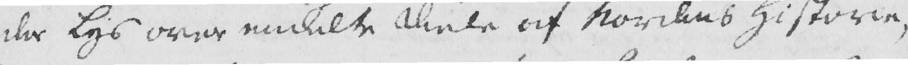der Lys over enkelte Deele af Nordens Historie,
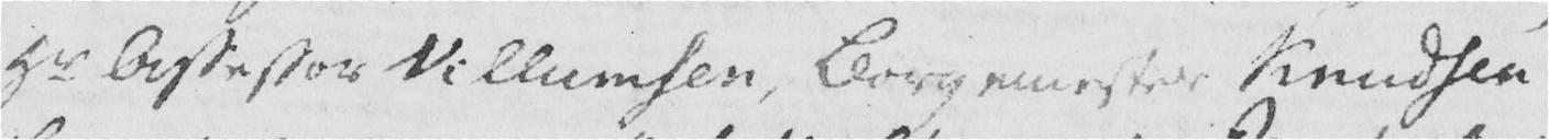Hr Assessor Villumsen, Borgemester Knudsen
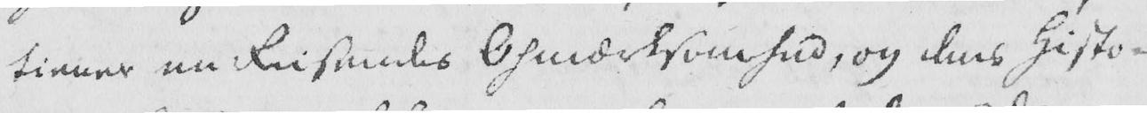tiener en Reisendes Opmærksomhed, og dens Histo-
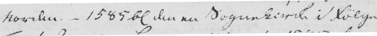Norden. - 1585 bl den en Sognekirke i Følge
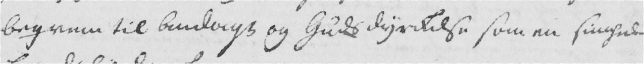beqvem til Andagt og Gudsdyrkelse som en simpel
